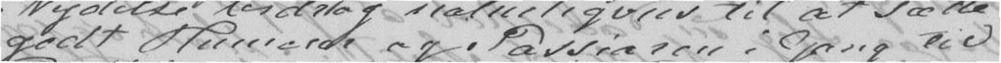godt Humerer og Passiaren i Gang til
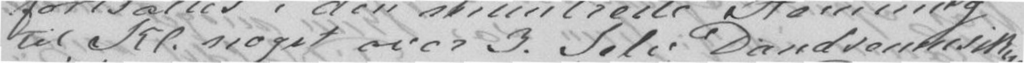til Kl. noget over 3. Selv Dandsemusiken
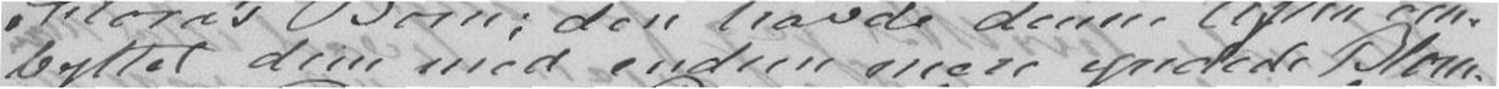byttet dem med endnu mere yndede Blom-
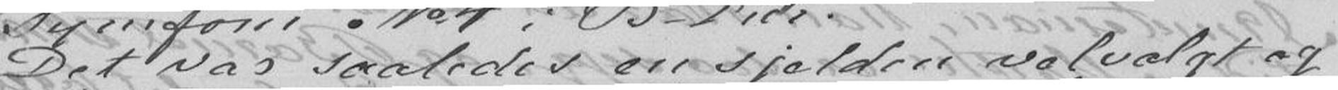Det var saaledes en sjelden velvalgt og
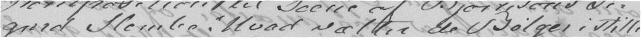gurd Slembe "Hvad vælter de Bølger i stille
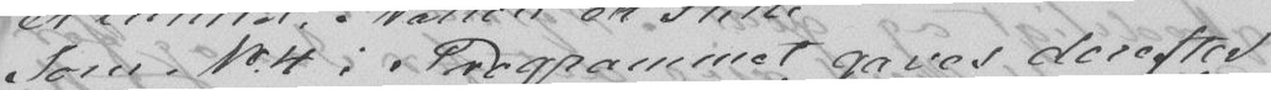Som N..4 i Programmet gaves derefter
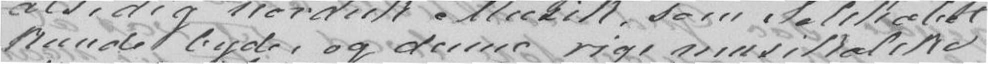kunde byde, og denne rige musikalske
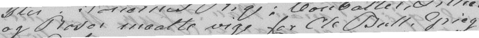og Roser maatte vige for Ole Bull, Grieg
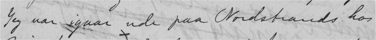Jeg var igaar ude paa Nordstrands hos
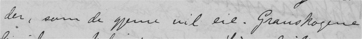der, som de gjerne vil eie. Granskogene
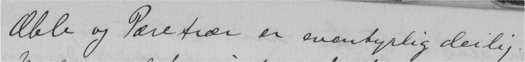Æble og Pæretrær er eventyrlig deilig.
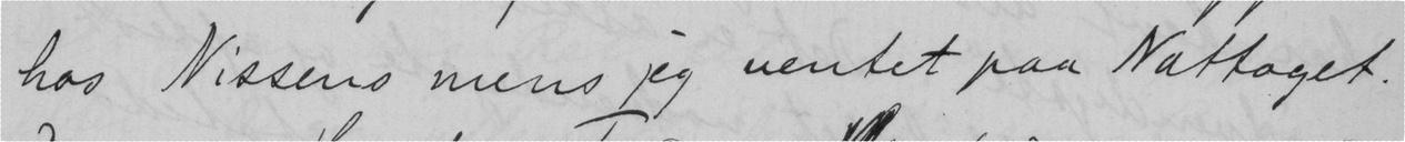hos Nissens mens jeg ventet paa Nattoget.
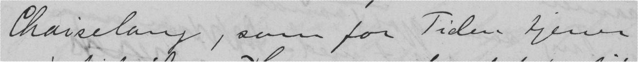Caiselong, som for Tiden tjener
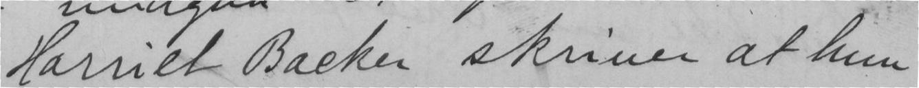Harriet Basker skriver at hun
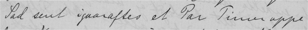Sad sent igaaraftes et Par Timer oppe
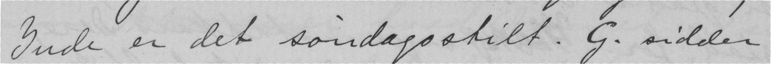Inde er det søndagsstilt. G. sidder
